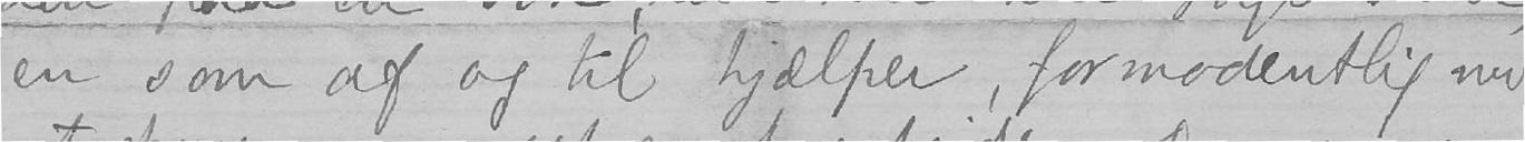en som af og til hjælper, formodentlig med
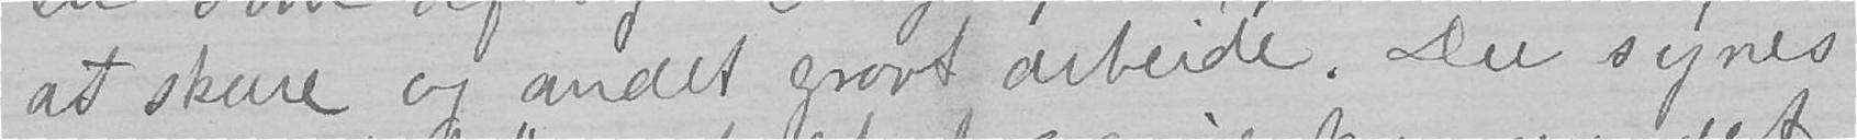at skure og andet grovt arbeidet. Du synes
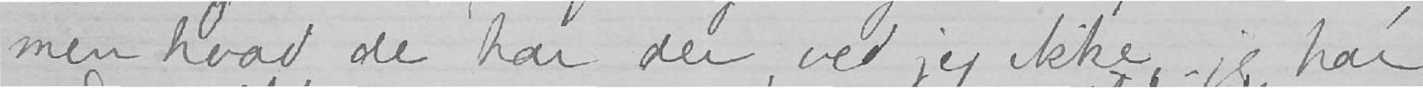men hva de har der, ved jeg ikke, jeg har
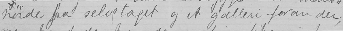høide fra selve taget og et galleri foran der,
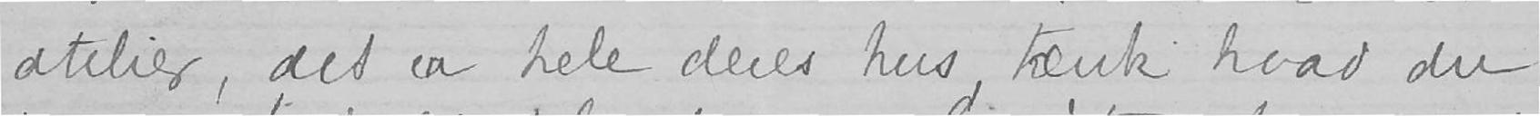atelier, det er hele deres hus, tænk hvad du
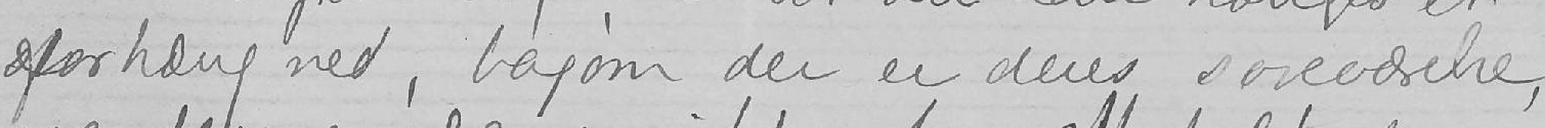forhæng ned, bagom der er deres soveværelse,
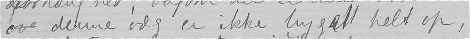ove denne væg er ikke bygget helt op,
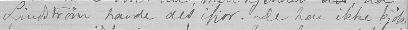Lindstrøm havde det ifjor. De har ikke kjøk-
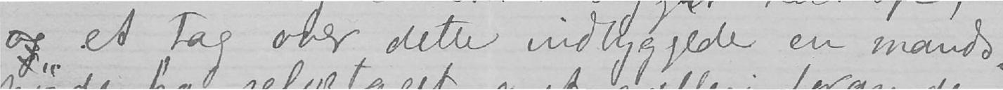og et tag over dette indbyggede en mands-
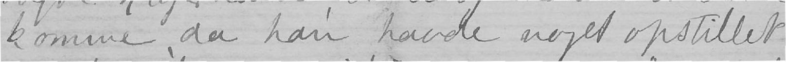komme, da han havde noget opstillet
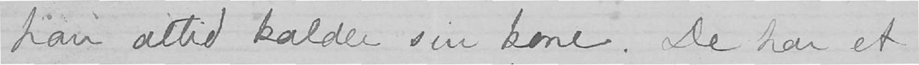han altid kalder sin kone. De har et
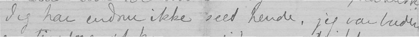Jeg har endnu ikke seet hende, jeg var buden
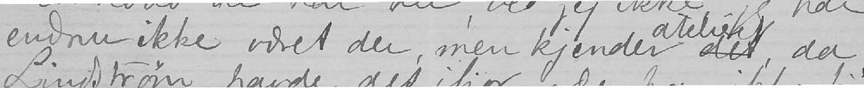endnu ikke været der, men kjender atelieret det,  da
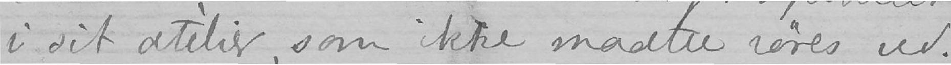i sit atelier, som ikke maatte røres ved.
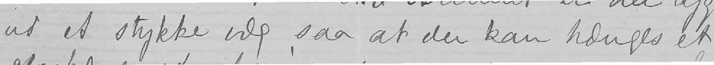ud et stykke væg, saa at der kan hænges et
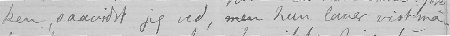ken, saavidst jeg ved, men hun laver vist ma-
